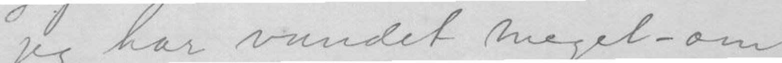jeg har vundet meget - om
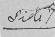Side 7
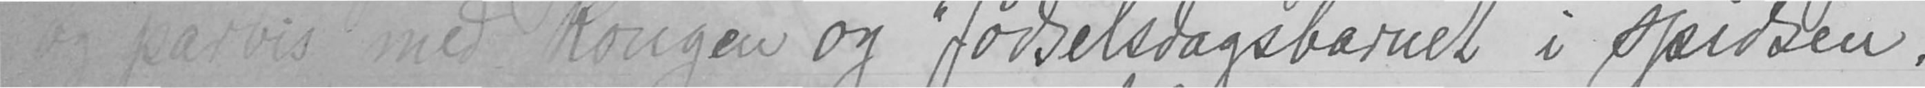og parvis med Kongen og "fødselsdagsbarnet" i spidsen.
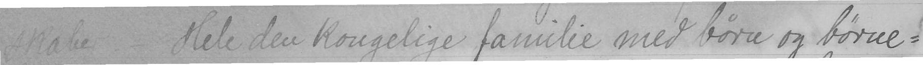skabe. - Hele den kongelige familie med børn og børne-
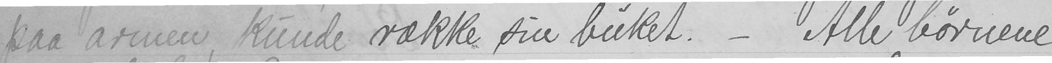paa armen, kunde række sin buket. - Alle børnene
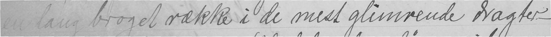en lang broget række i de mest glimrende dragter, -
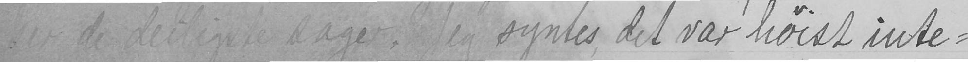ser de deiligste sager. Jeg syntes, det var høist inte-
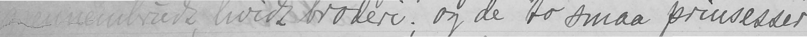gjennembruds, hvidt broderi; og de to smaa prinsesser
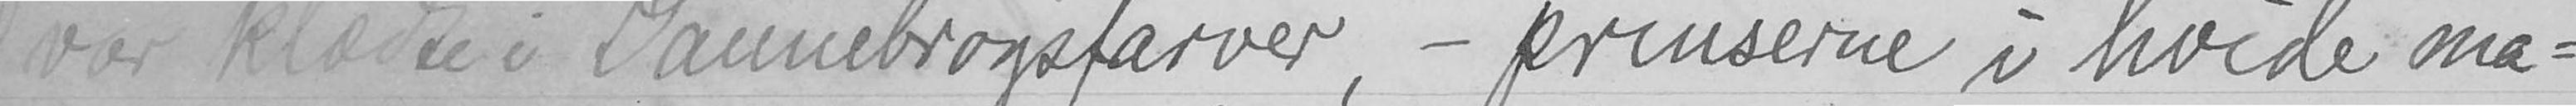var klæde i Dannebrogsfarver, - prinserne i hvide ma-
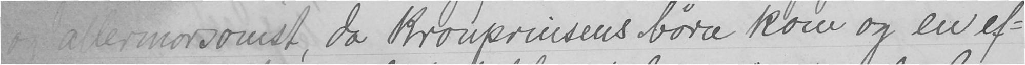og aller morsomst, da kronprinsens børn kom og en ef-
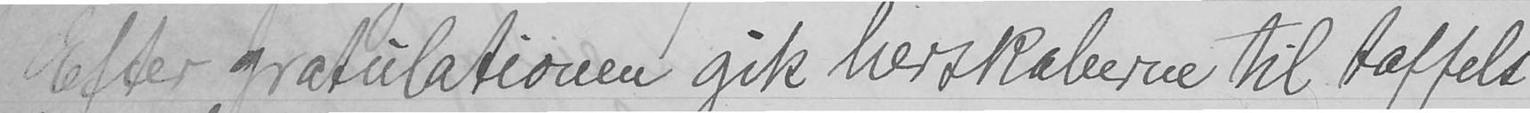Efter gratulationen gik herskaberne til taffels
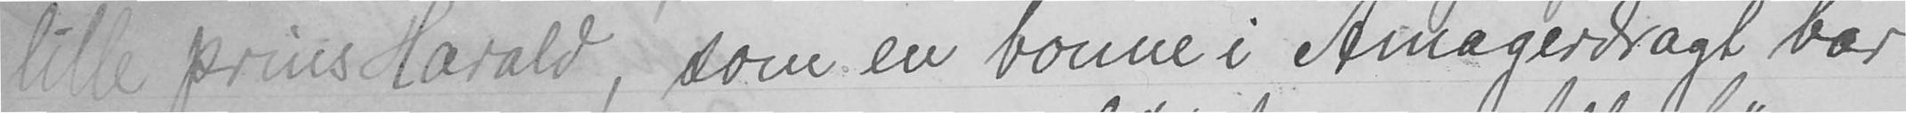lille prins Harald, som en bonne i Amagerdragt bar
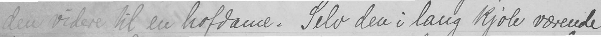den videre til en hofdame. Selv den i lang kjole værende
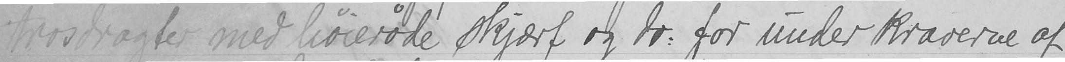trosdragte med høierøde skjerf og dr: for under kraverne af
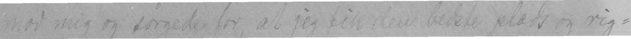mod mig og sørgede for at jeg fik den bedste plads og rig-
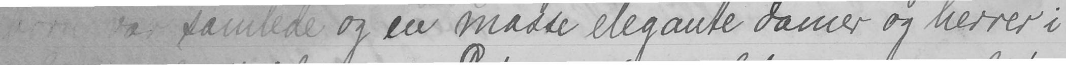børnene samlede og en masse elegante damer og herrer i
